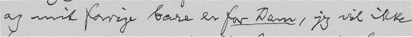og mit forrige bare er for Dem, jeg vil ikke
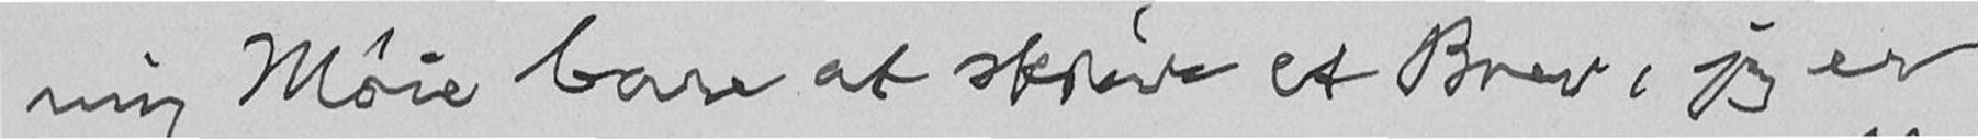mig Møie bare at skrive et Brev, jeg er
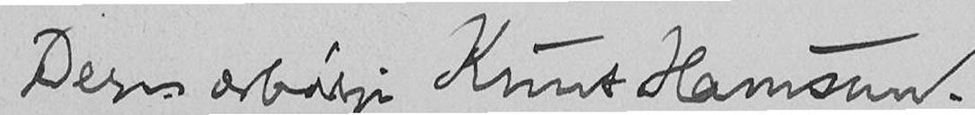Deres ærbødige Knut Hamsun.
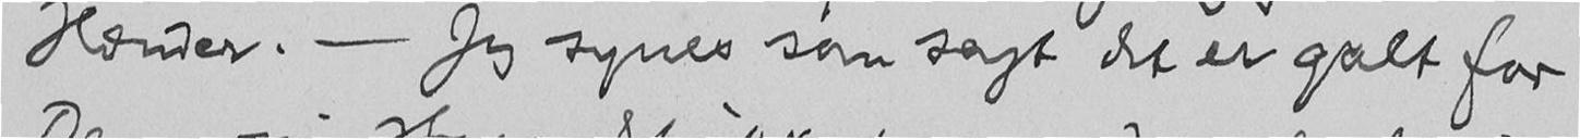Hænder. - Jeg synes som sagt det er galt for
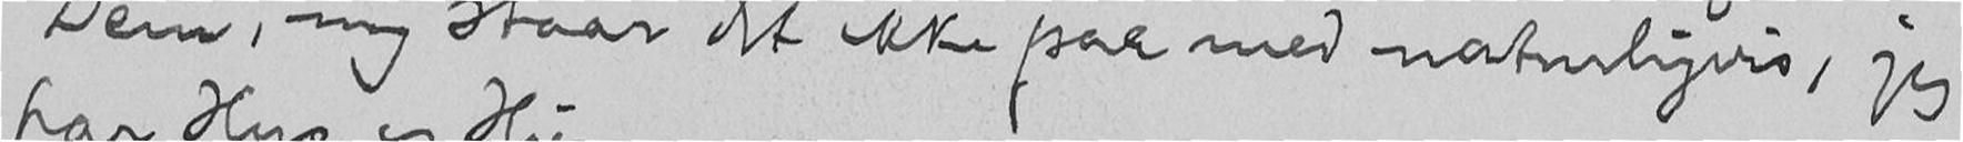Dem, mig staar det ikke paa med naturligvis, jeg
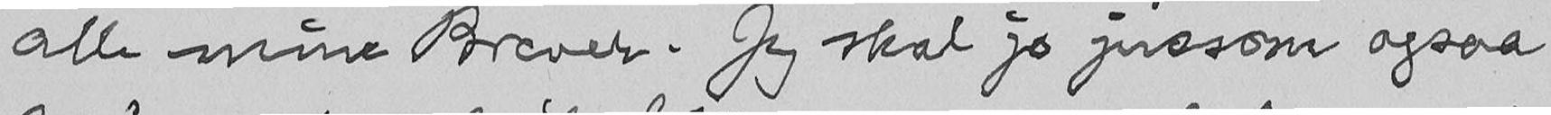alle mine Brever. Jeg skal jo jussom ogsaa
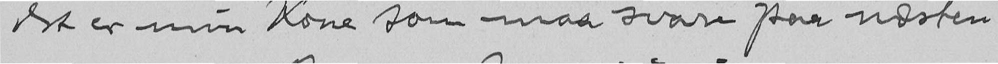det er min Kone som maa svare paa næsten
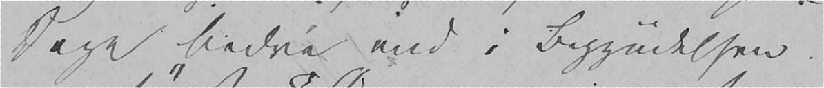Dage bedre end i Begyndelsen.
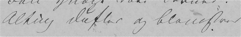Alting dufter og blomstrer
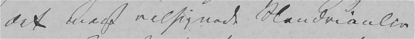det meest velsignede Slendrianliv
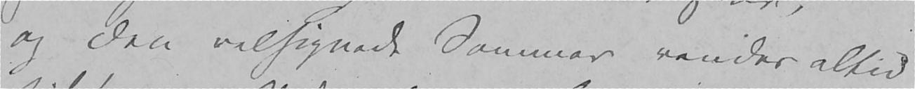og den velsignede Sommer vender altid
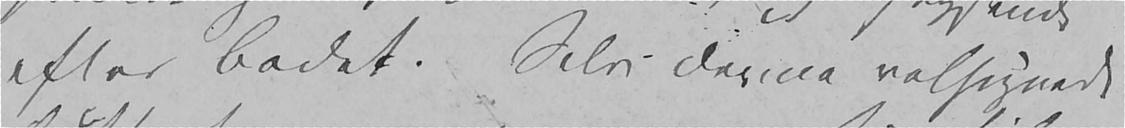efter Badet. Selv denne velsignede
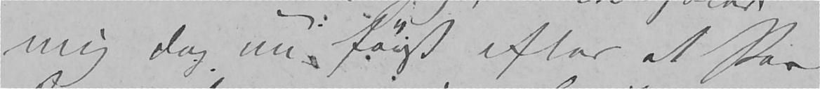mig dog nu først efter et Par
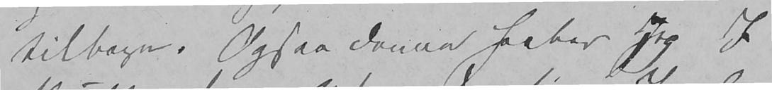tilbage. Ogsaa denne haaber jeg I
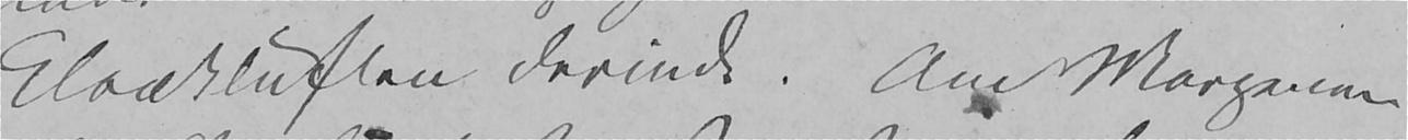Kloakluften derinde. Om Morgenen
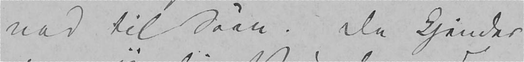ned til Søen. Du kjender
